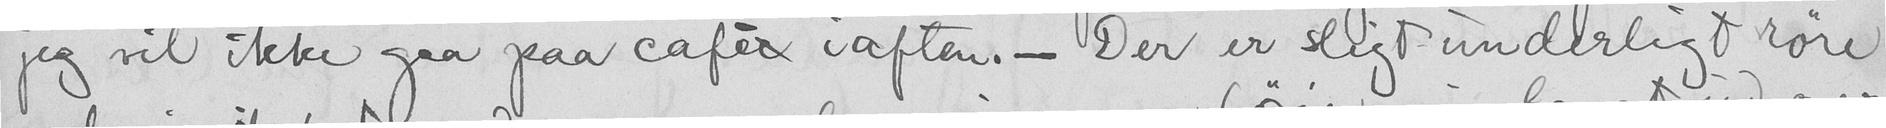jeg vil ikke gaa paa café iaften. - Der er sligt underligt røre
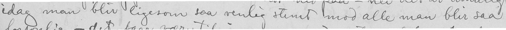idag man blir ligesom saa venlig stemt mod alle man blir saa
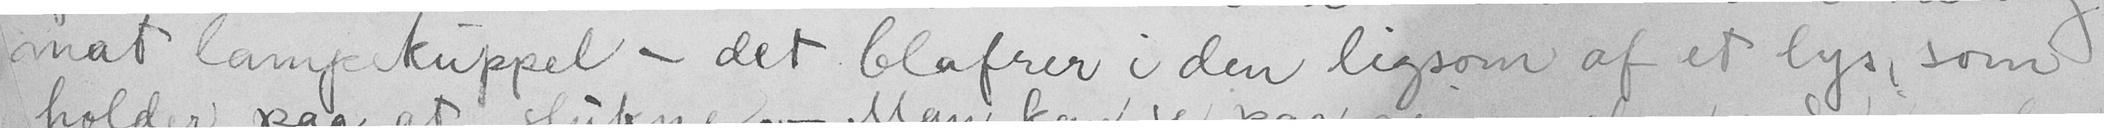mat lampkuppel, - det blafrer i den ligsom af et lys, som
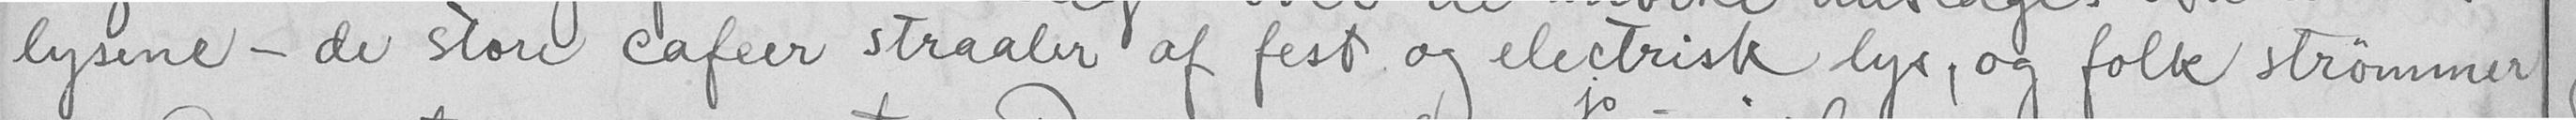lysene - de store cafeer straaler af fest og electrisk lys, og folk strømmer
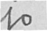jo
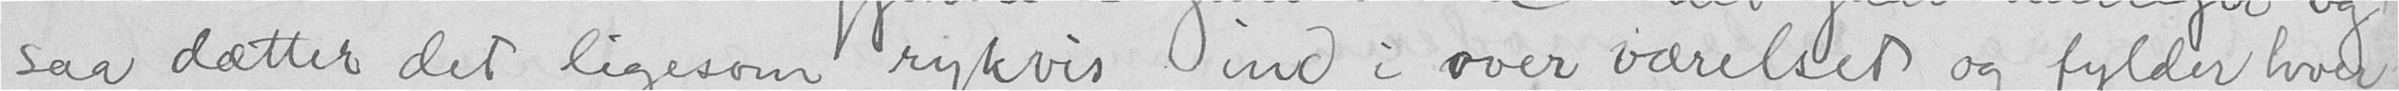saa dætter det ligesom rykvis ind i over værelset og fylder hver
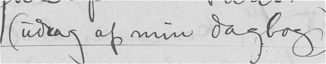(udrag af min dagbog)
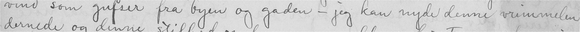vind som gufser fra byen og gaden - jeg kan nyde denne vrimmelen
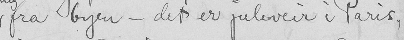fra byen - det er juleveir i Paris,
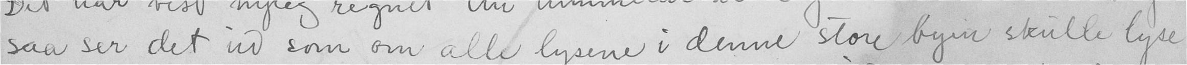saa ser det ud som om alle lysene i denne store byen skulle lyse
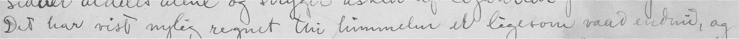Det har vist nylig regnet tihi himmelen et ligesom vaad endnu, og
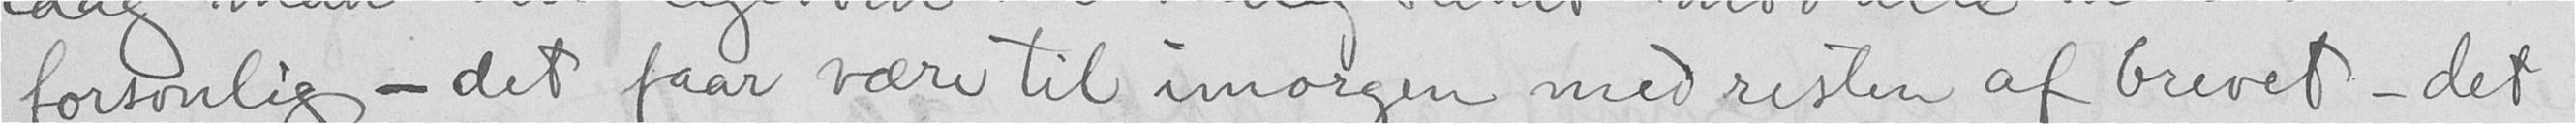forsonlig - det faar være til imorgen med resten af brevet - det
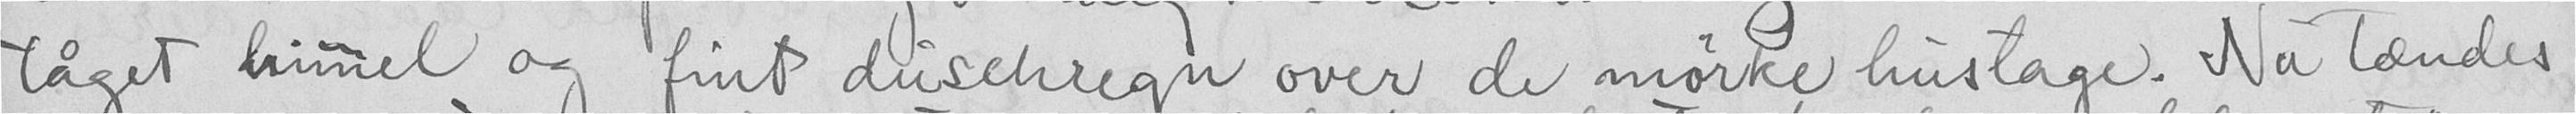tåget himel og fint duschregn over de mørke hustage. Nu tændes
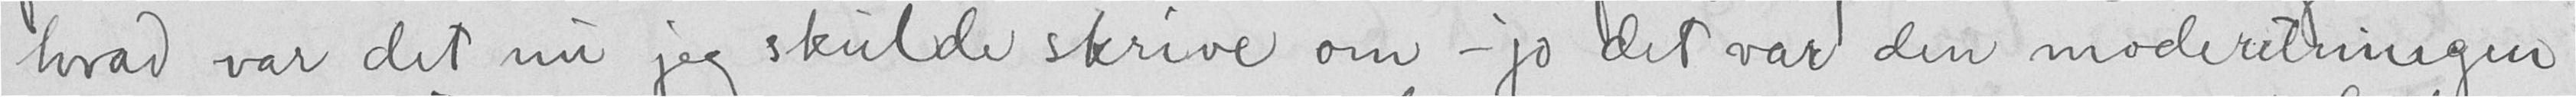hvad var det nu jeg skulde skrive om - jo det var den moderetningen
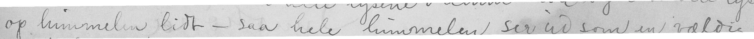op himmelen lidt - saa hele himmelen ser ud som en vældig
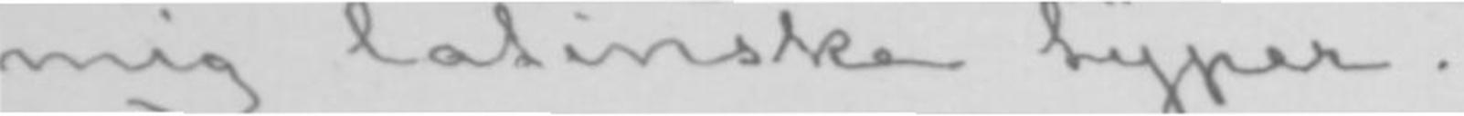mig latinske typer.
